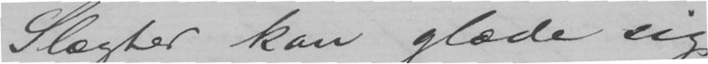Slægter kan glæde sig
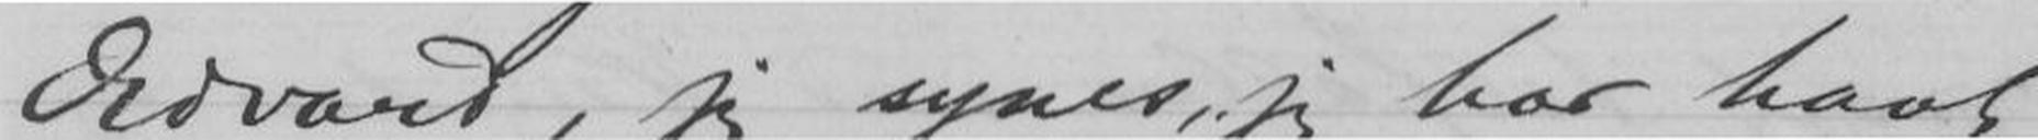Edvard, jeg synes jeg har havt
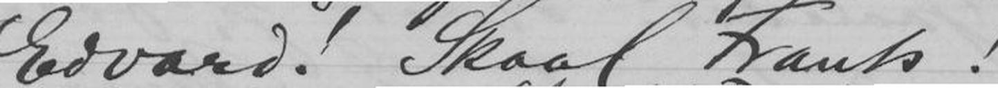Edvard! Skaal Frants!
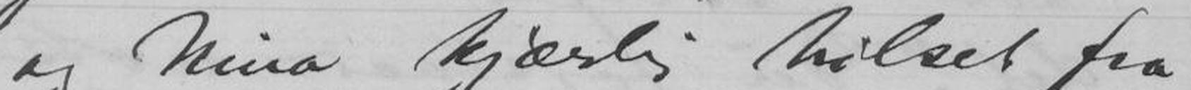og Nina kjærlig hilset fra
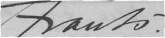Frants.
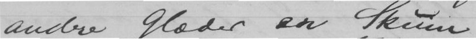andre Glæder er Skum
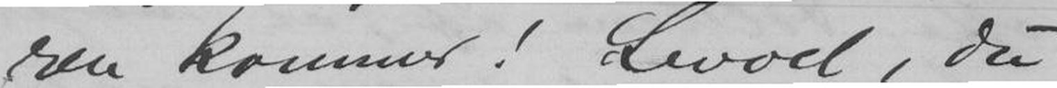ren kommer! Levvel, Du
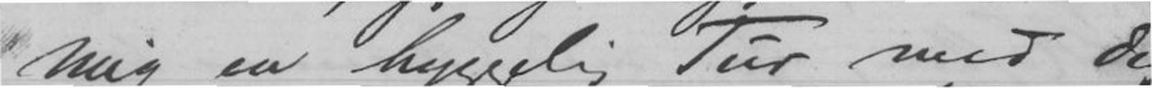mig en hyggelig Tur med Dig,
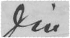Din
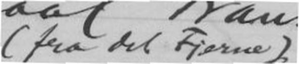(fra det Fjerne)
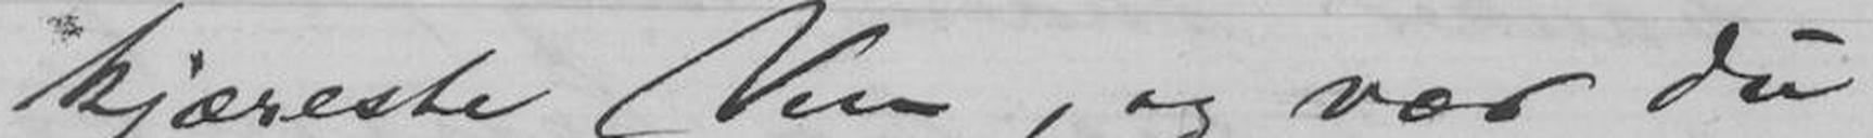kjæreste Ven, og vær Du
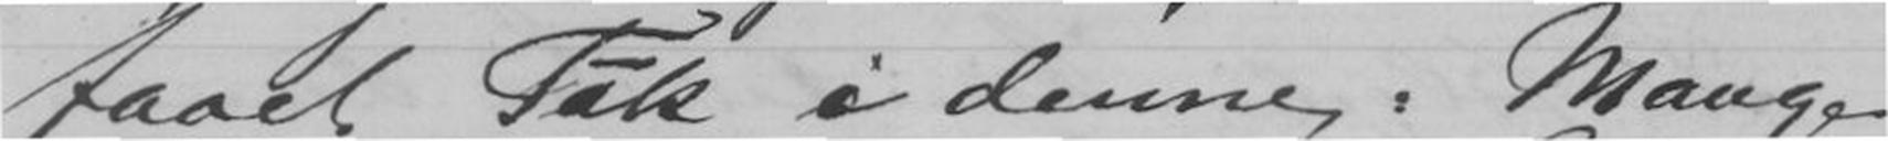faaet Ták i denne. Mange
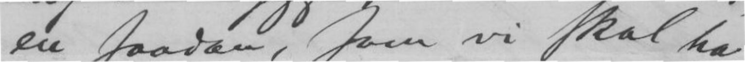en saadan, som vi skal ha
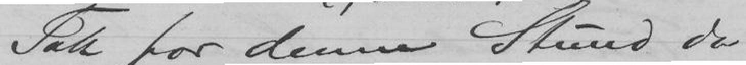Tak for denne Stund da
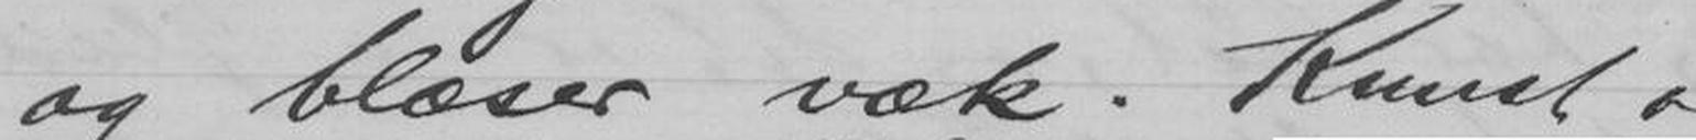og Blæser væk. Kunst og
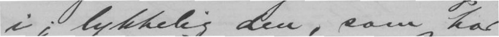i; lykkelig den, som har
